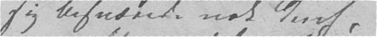sig besnærede nok deraf,
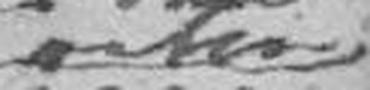rette
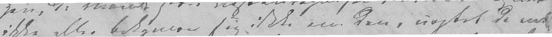ikke eller bekymre sig ikke om den, uagtet de endog
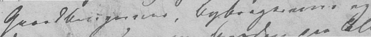Gaardbrugerens, Byborgerens og
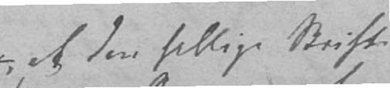at den hellige Skrift
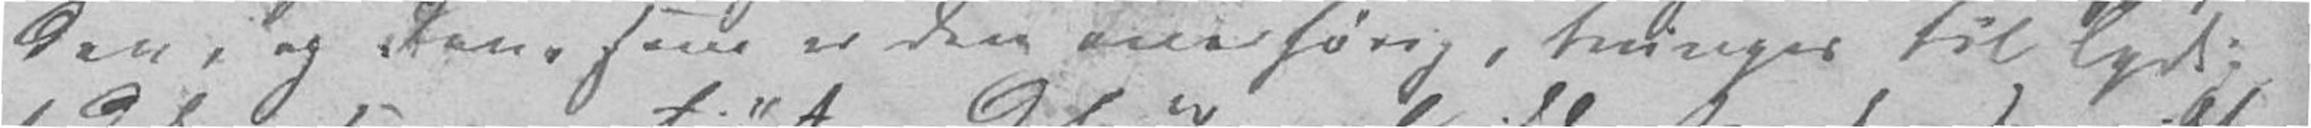den, og den som er den ene hørig, tvinges til Lydig-
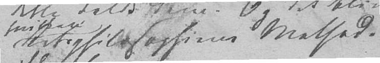Retsphilosophiens Methode
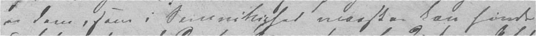er dem, som i Samvittighed maaske kan finde
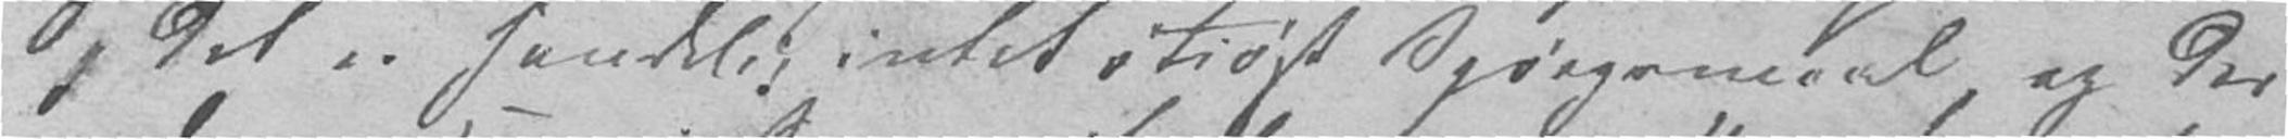Og det er sandelig intet otiøst Spørgsmaal og der
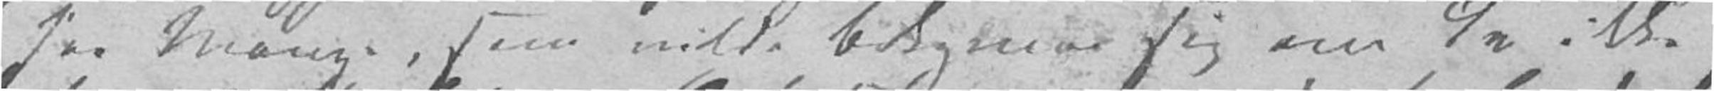saa Mange, som vilde bekymre sig om de ikke
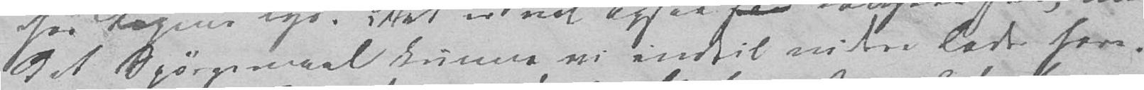det Spørgsmaal kunne vi indtil videre lade fare.
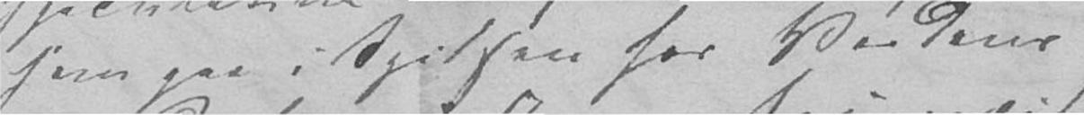som gaa i Spidsen for Verdens
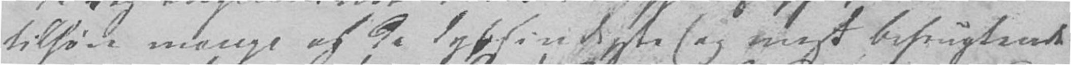tilhøre mange af de Dybsindigste og meest befrugtende
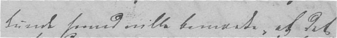kunde hermed ville bemærke, at det
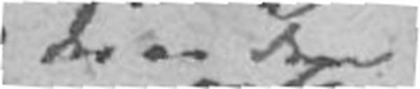der er den
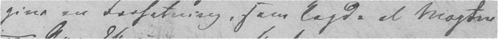give en Forfatning, som lagde al Magten
